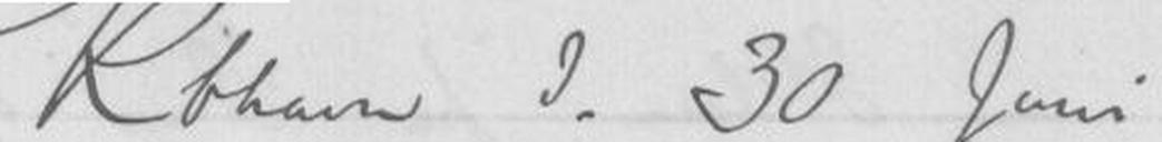Kbhavn d. 30 Juni
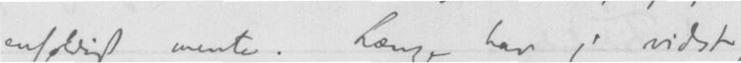enfoldig mente. Længe har jeg vidst,
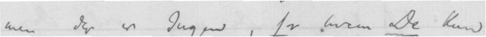men der er Ingen, for hvem De kun
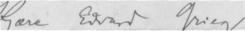Kjære Edvard Grieg
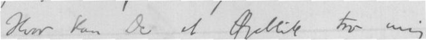Hvor kan De et Øjeblik tro mig
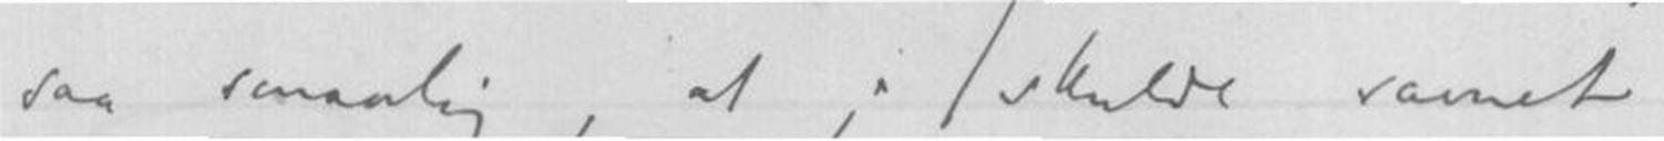saa smaalig, at jeg skulde savnet
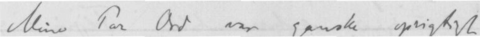Mine Par Ord var ganske oprigtigt
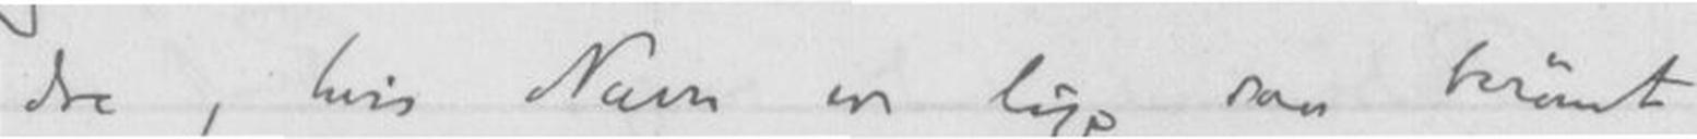dre, hvis Navn er lige saa berømt,
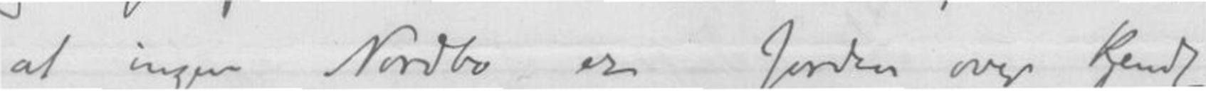at ingen Nordbo er Jorden over kjendt
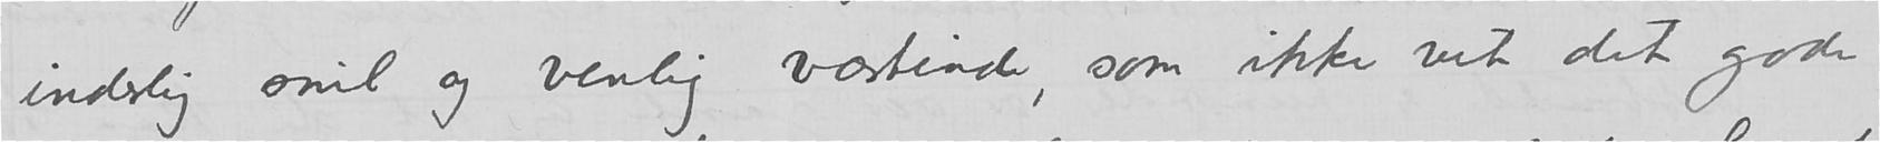inderlig snil og venlig værtinde, som ikke vet det gode
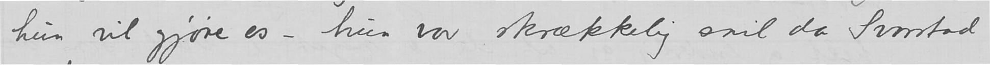hun vil gjøre os – hun var skrækkelig snil da Svarstad
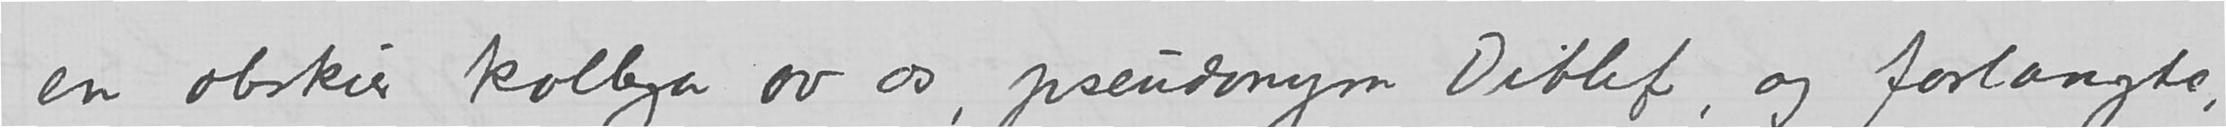en obskur kollega av os, pseudonym Ditlef, og forlangte,
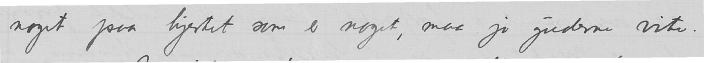noget paa hjertet som er noget, maa jo guderne vite. –
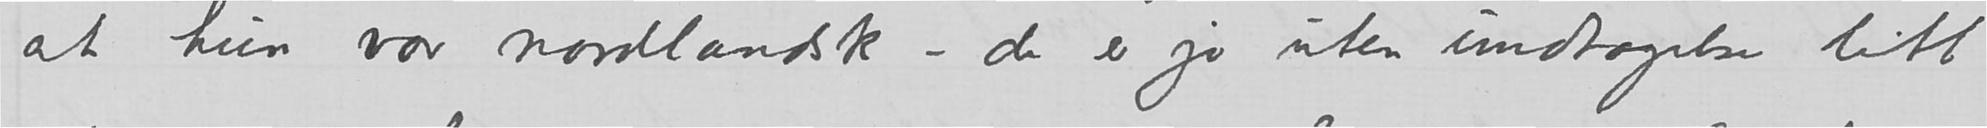at hun var nordlandsk – de er jo uten undtagelse litt
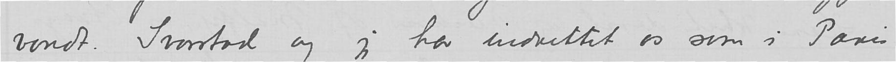vondt. Svarstad og jeg har indrettet os som i Paris
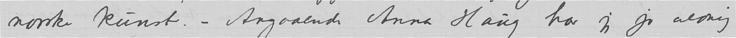norske kunst. – Angaaende Anna Hang har jeg jo aldrig
